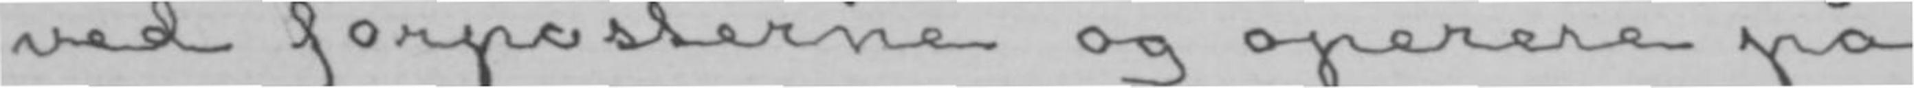ved forposterne og operere på
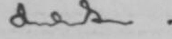det.
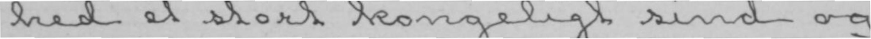hed et stort kongeligt sind og
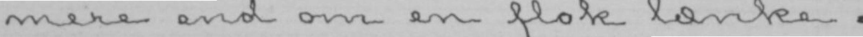mere end om en flok lænke-
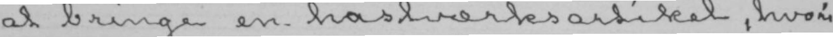at bringe en hastværksartikel, hvori
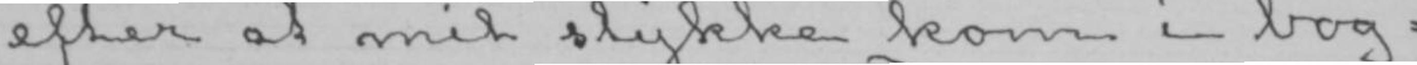efter at mit stykke kom i bog-
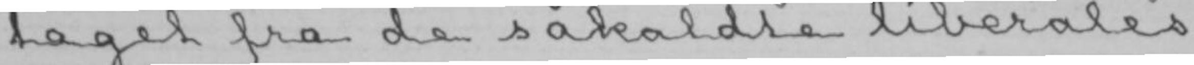taget fra de såkaldte liberales
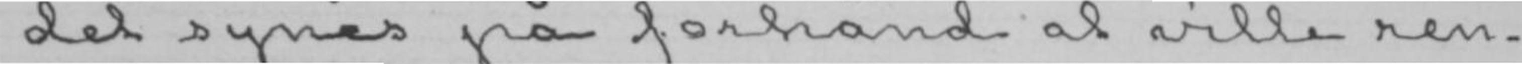det synes på forhånd at ville ren-
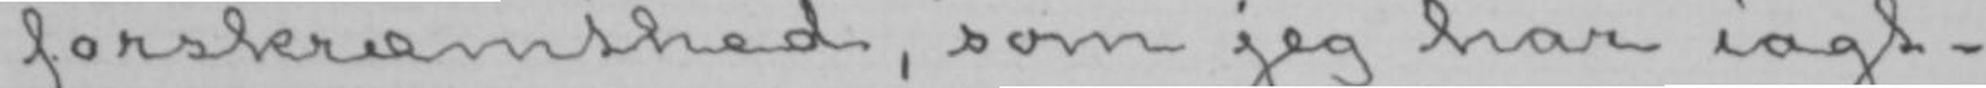forskræmthed, som jeg har iagt-
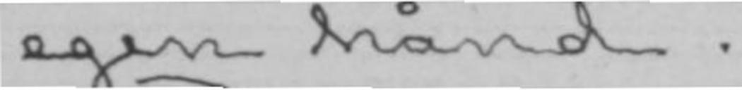egen hånd.
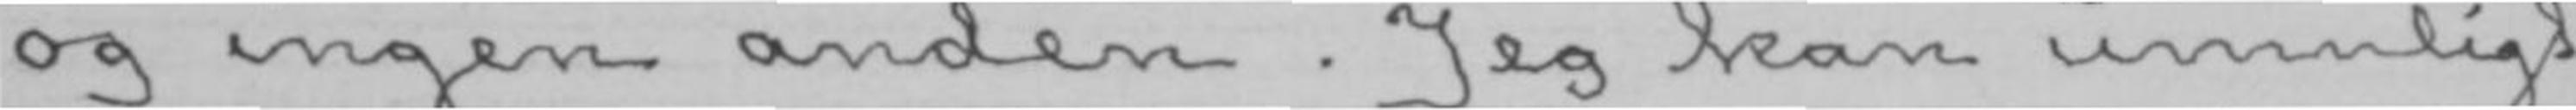og ingen anden. Jeg kan umuligt
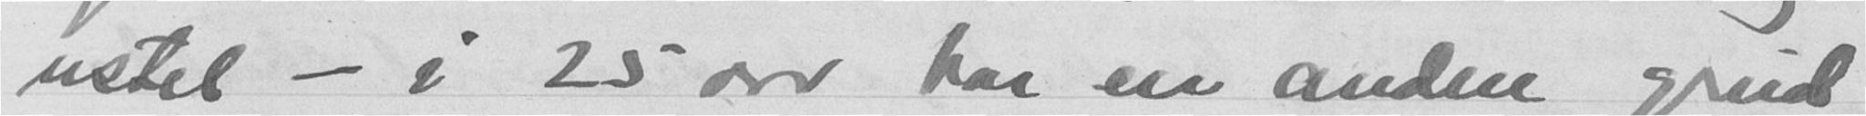ustel - i 25 aar har en anden greid
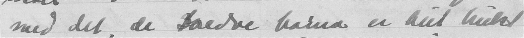med det, de bedre barna er blit brukt
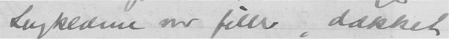Sengklærne var filler, dækket
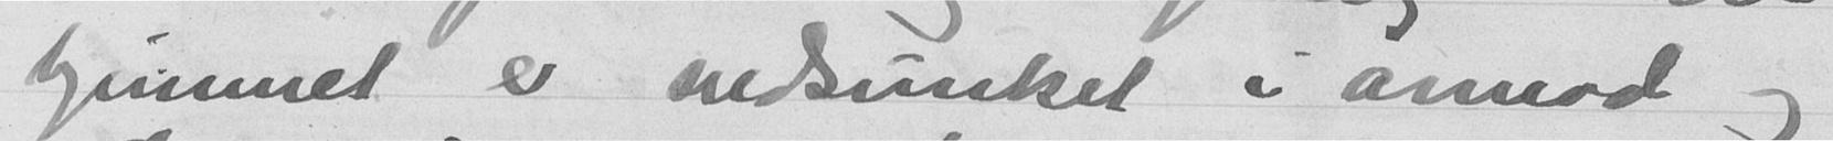hjemmet er nedsunket i armod og
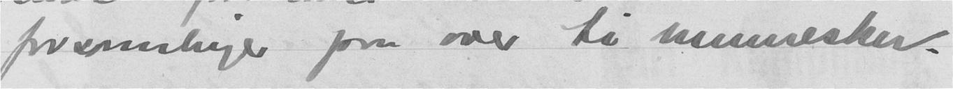forsamlinger paa over ti mennesker.
